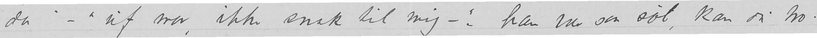da» -« uf mor, ikke snak til mig - « , han var saa søt, kan du tro.
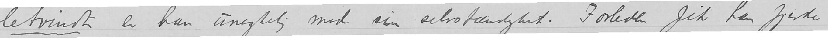letvindt er han unegtelig med sin selvstændighet. Forleden fik han fjerde
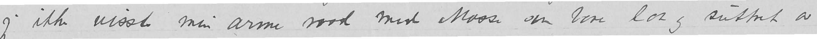jeg ikke visste min arme raad med Mosse som bare laa og suttret av
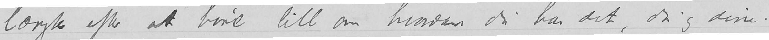længter efter at høre litt om hvordan du har det, du og dine.
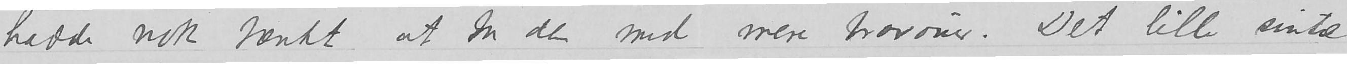hadde nok tænkt at ta den med mere bravour. Det lille sinte
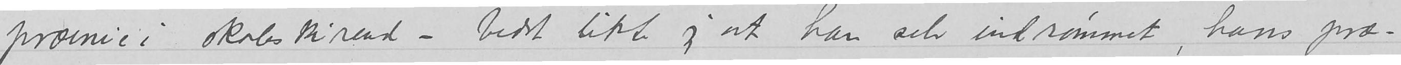præmie i skoleskirend – bedst likte jeg at han selv indrømmet, hans præ
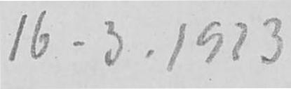16-3-1923
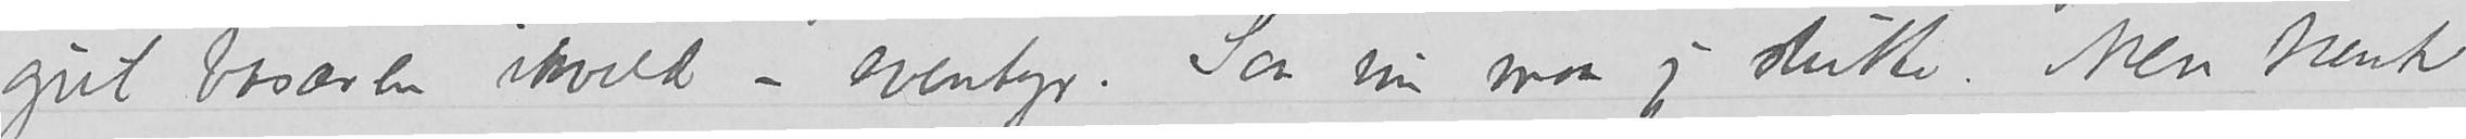gutbasaren ikveld - eventyr. Saa nu maa jeg slutte. Men tenk
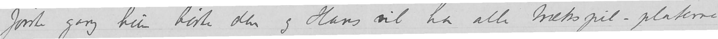første gang hun hørte den og Hans vil ha alle trækspil-platerne,
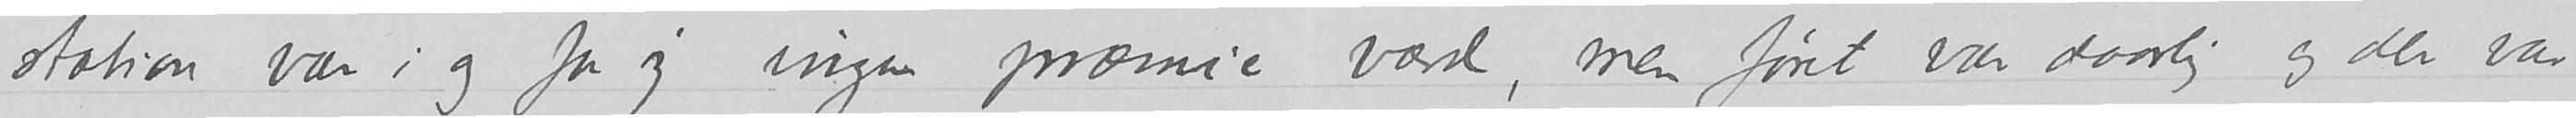station var i og for sig ingen præmie værd, men føret var daarlig og der var
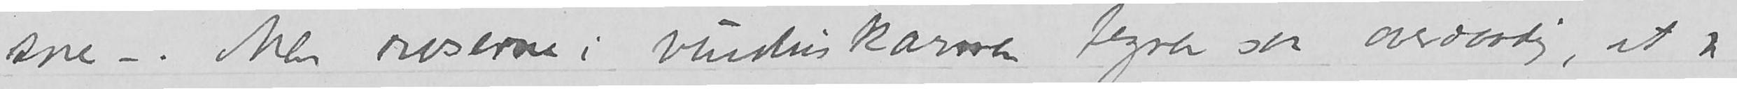sne –. Men roserne i vinduskarmen tegner saa overdaadig, at jeg
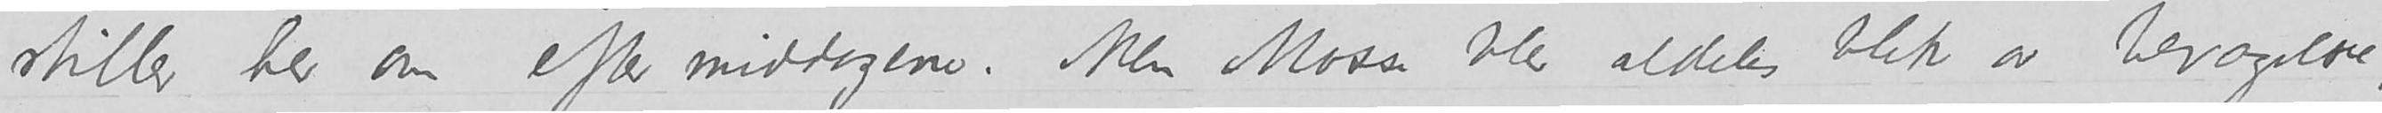stiller her om eftermiddagene. Men Mosse blev aldeles blek av bevægelse,
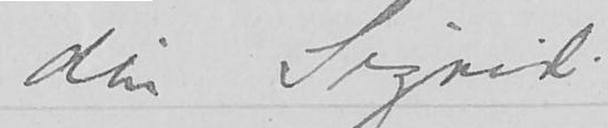din Sigrid.
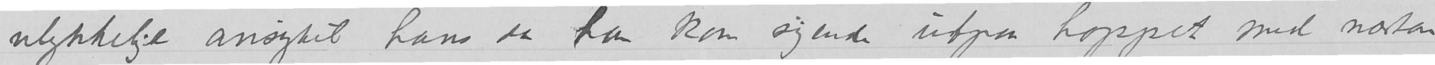ulykkelige ansigtet hans da han kom sigende utpaa hoppet med næsten
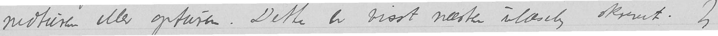nedturen eller opturen. Dette er visst næsten ulæselig skrevet. Jeg
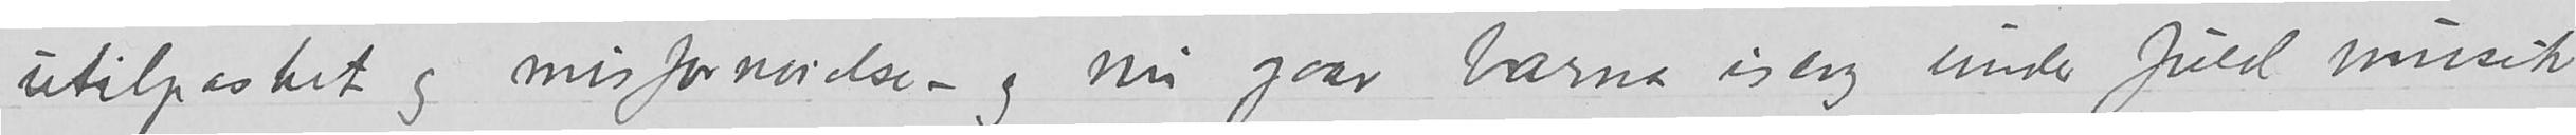utilpashet og misfornøielse – og nu gaar barna iseng under fuld musik
